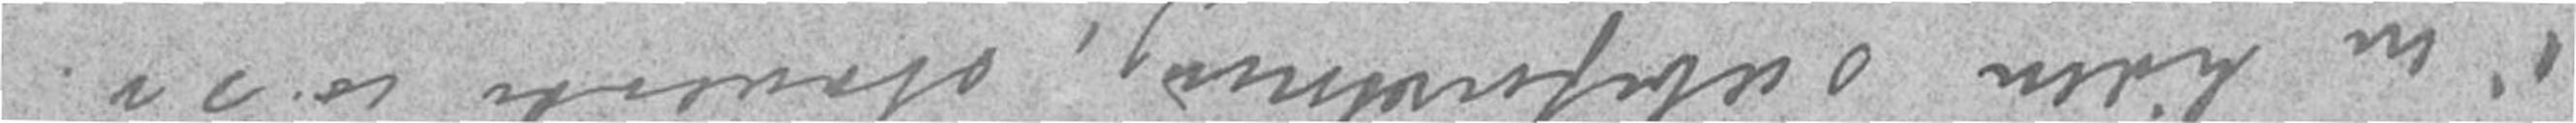Undskyld især konvoluten, jeg maatte expedere det
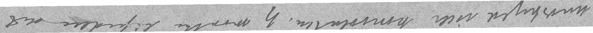i en liden sæbeforetning, staaende o.s.v.
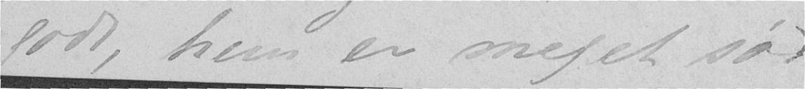godt, hun er meget sød
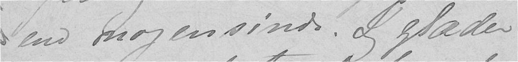end nogensinde. Jeg glæder
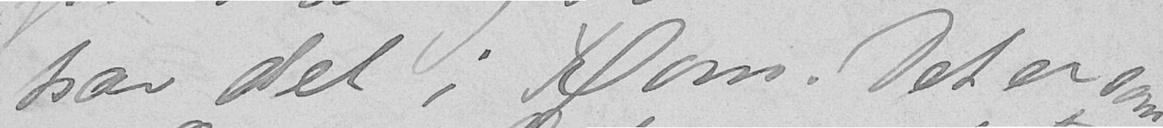har det i Rom. Det er som
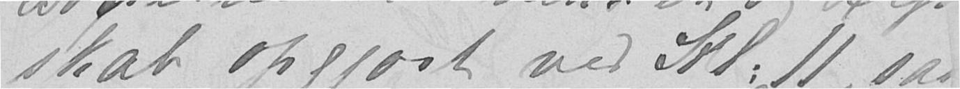skab opgjort ved Kl: 11, saa
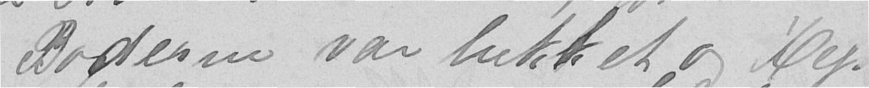Boderne var lukket og Rep-
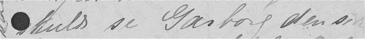skulde se Garborg den sid
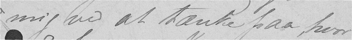mig ved at tænke paa, hvor
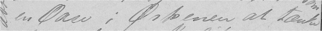en Oase i Ørkenen at tænke
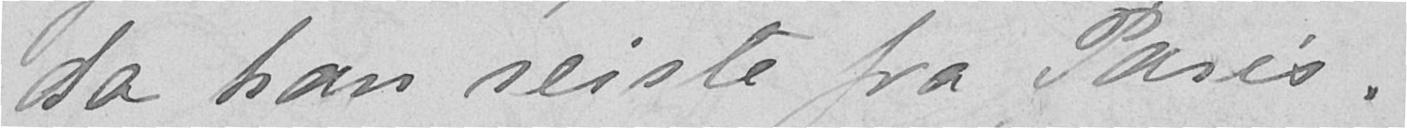da han reiste fra Paris.
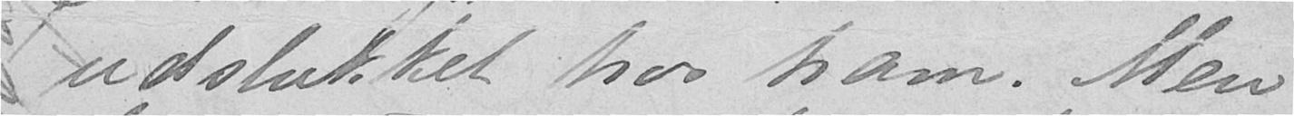udslukket hos ham. Men
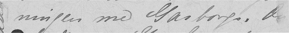ningen med Garborgs. Du
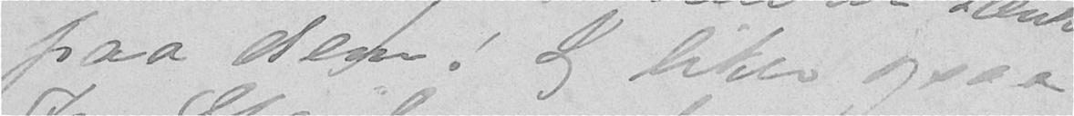paa dem! Jeg liker ogsaa
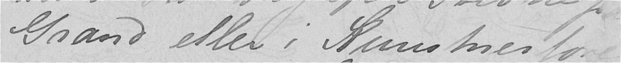Grand eller i Kunstnerfore-
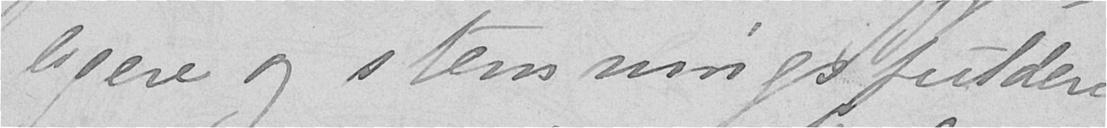ligere og stemningsfuldere
